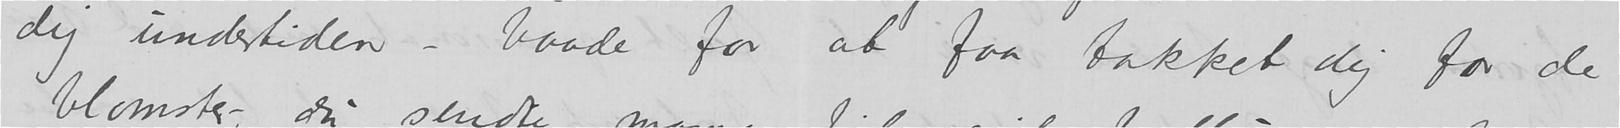dig undertiden – baade for at faa takket dig for de
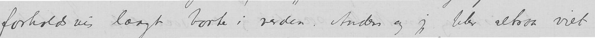forholdsvis langt borte i verden. Anders og jeg blev altsaa viet
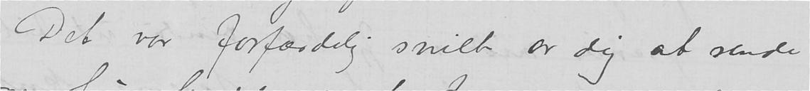Det var forfærdelig snilt av dig at sende
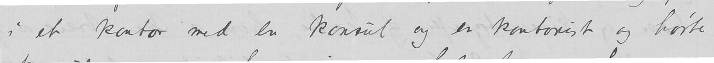i et kontor med en konsul og en kontorist og hørte
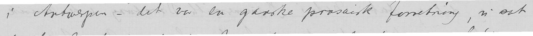i Antwerpen – det var en ganske prossaisk forretning, vi sat
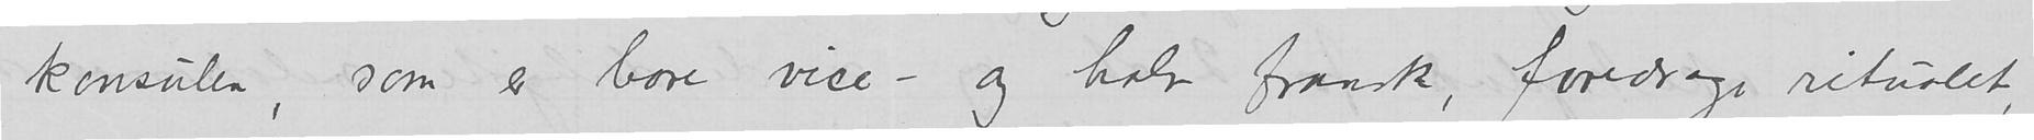konsulen, som er bare vice– og halv fransk, foredrage ritualet,
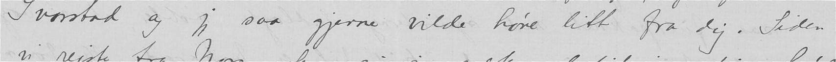Svarstad og jeg saa gjerne vilde høre litt fra dig. Siden
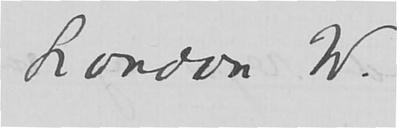London W.
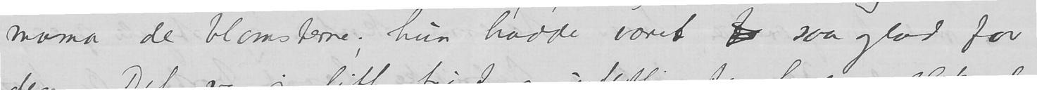mama de blomsterne hun hadde været for saa glad for
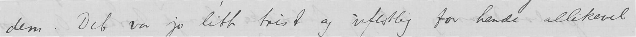dem. Det var jo litt trist og ufestlig for hende allikevel
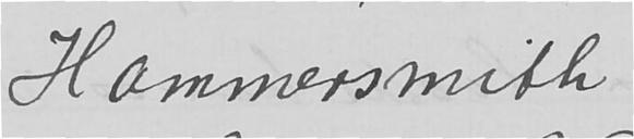Hammersmith
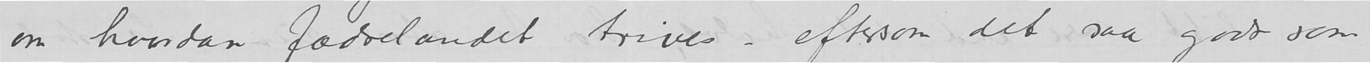om hvordan fædrelandet trives – eftersom det saa godt som
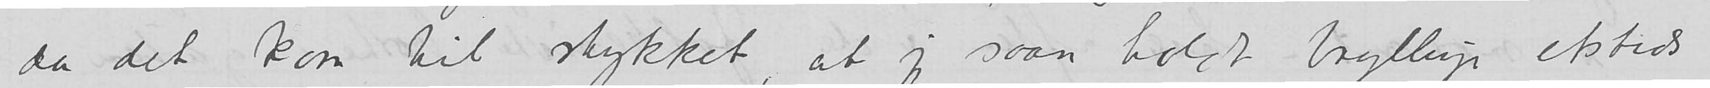da det kom til stykket, at jeg saan holdt bryllup etsteds
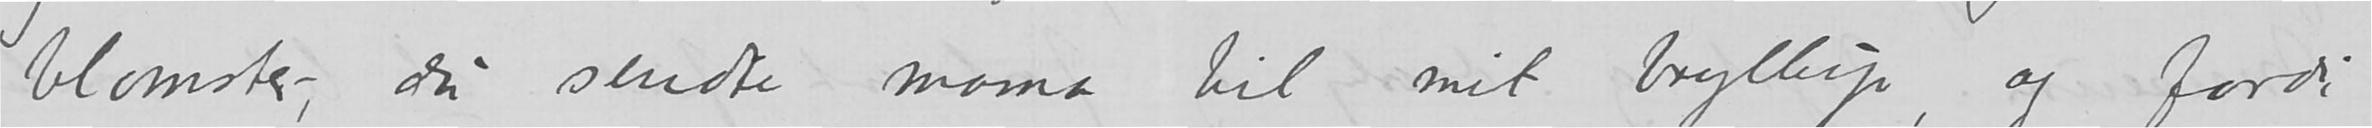blomster, du sendte mama til mit bryllup, og fordi
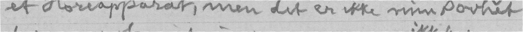et Høreapparat, men det er ikke min Døvhet
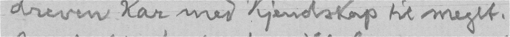dreven Kar med Kjendskap til meget.
